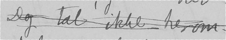Dog tal ikke herom
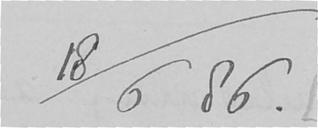18/6 86.
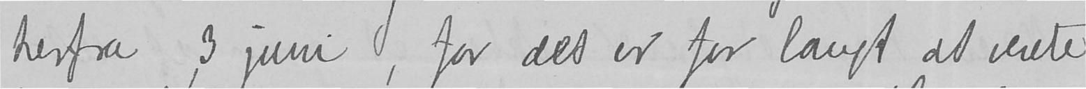herfra 3 juni, for det er for langt at vente
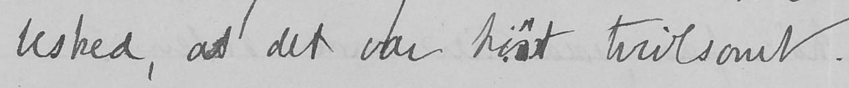besked, at det var høist tvivlsomt.
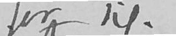for dig.
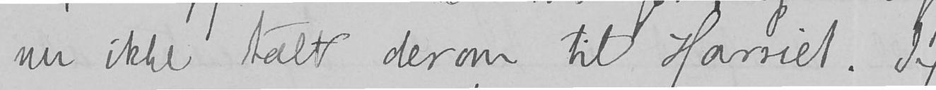nu ikke talt derom til Harriet. Jeg
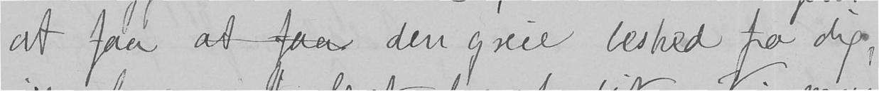at faa at faa den greie besked fra dig,
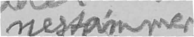nestammer
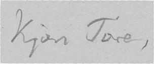Kjære Tore,
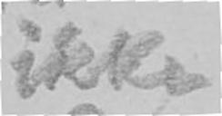ikke
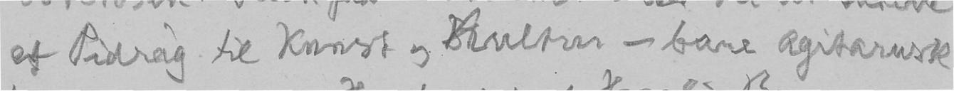et Pidrag til Kunst og Kultur - bare agitarusk
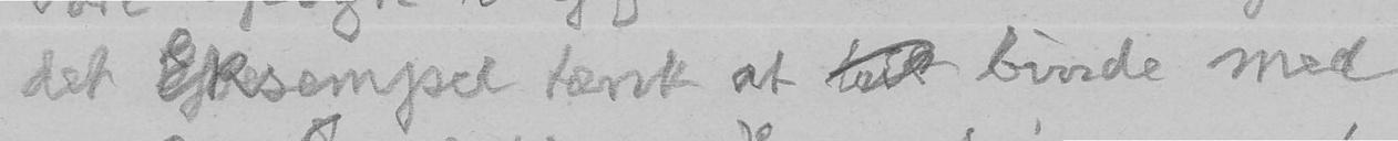det Eksempel tænk at læse binde med
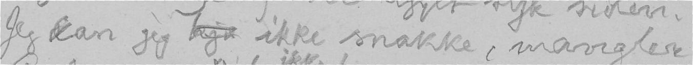Jeg kan jeg kje ikke snakke, mangler
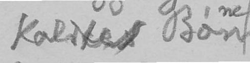kalder Bøn
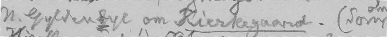N. Gyldendyl om Kierkegaard. (Som
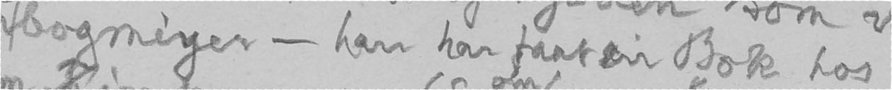bogmeyer - han har faat en Bok hos
